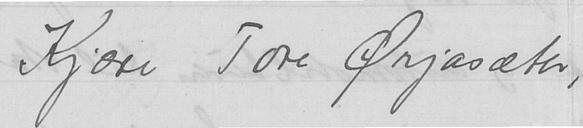Kjære Tore Ørjasæter,
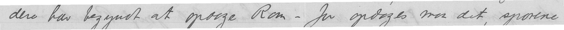dere har begyndt at opdage Rom – for opdages maa det, sporene
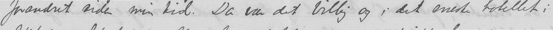forandret siden min tid. Da var det billig og i det eneste hotellet i
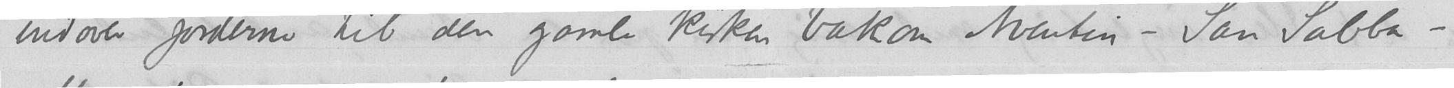indover jorderne til den gamle kirken bakom Aventin – San Sabba –
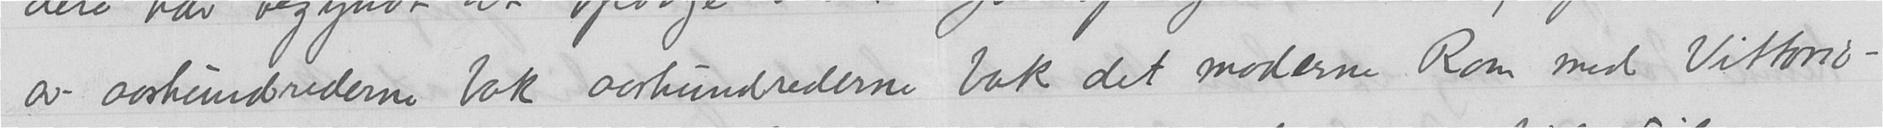av aarhundrederne bak aarhundrederne bak det moderne Rom med Vittorio-
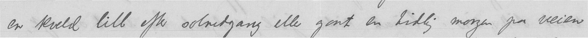en kveld litt efter solnedgang eller gaat en tidlig morgen paa veien
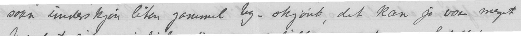saan underskjøn liten gammel by - skjønt, det kan jo være meget
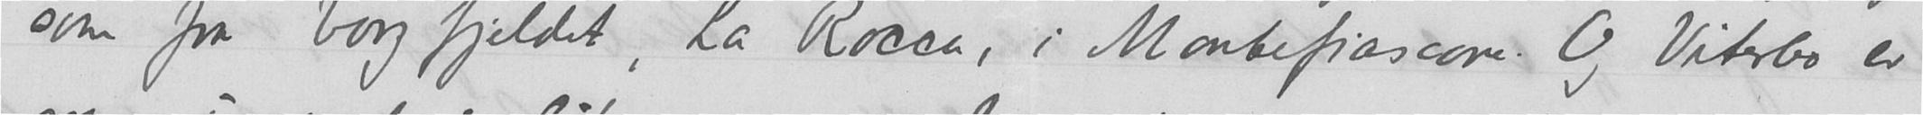som fra borgfjeldet, La Rocca, i Montefiascone. Og Viterbo er
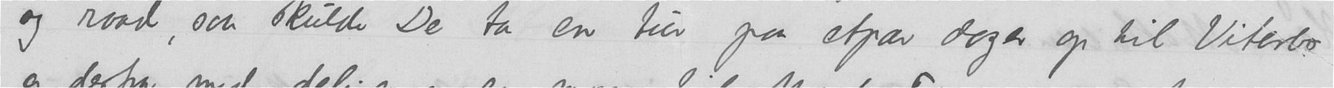og raad, saa skulde De ta en tur paa etpar dager op til Viterbo
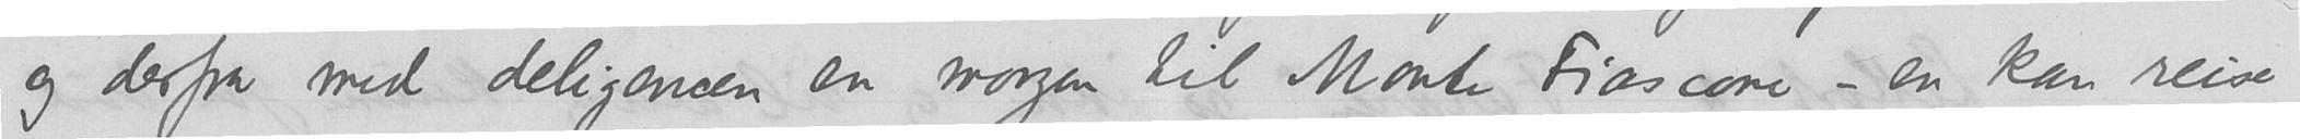og derfra med deligencen en morgen til Monte Fiascone – en kan reise
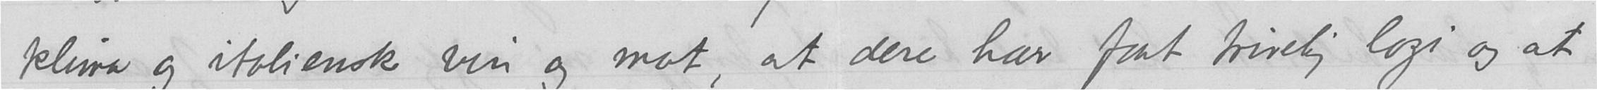klima og italiensk vin og mat, at dere har faat trivelig logi og at
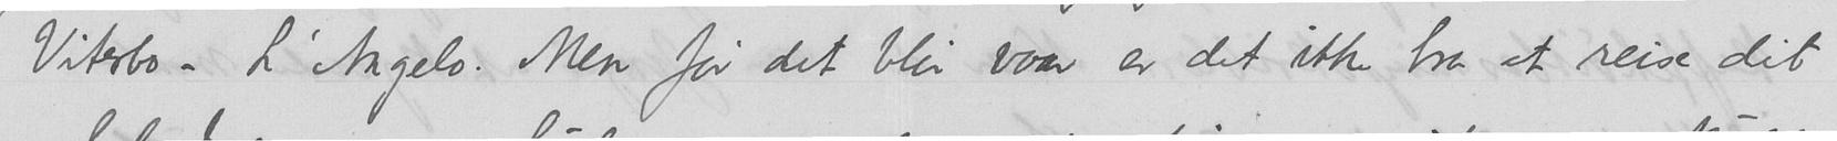Viterbo – L’ Angelo. Men før det blir vaar er det ikke bra et reise dit
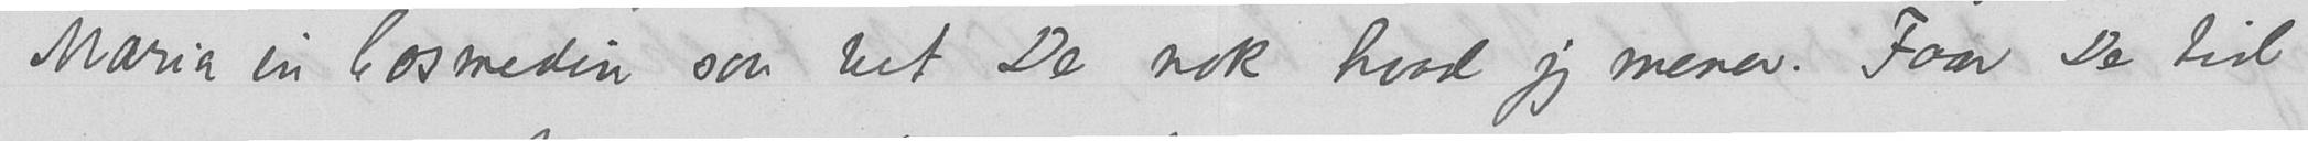Maria in Cosmedin saa vet De nok hvad jeg mener. Faar De tid
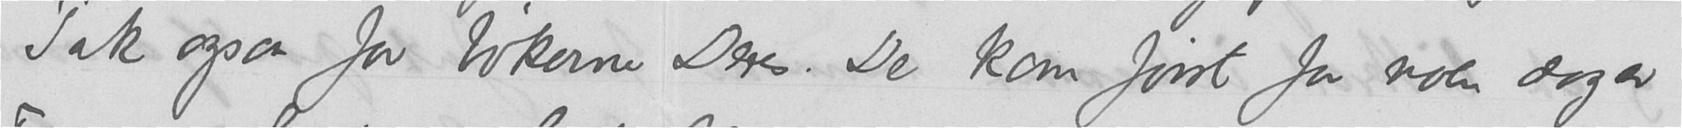Tak ogsaa for bøkerne Deres. De kom først for noen dager
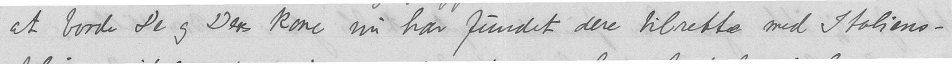at baade De og Deres kone nu har fundet dere tilrette med Italiens-
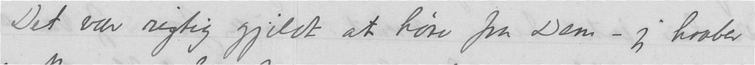Det var rigtig gjildt at høre fra Dem – jeg haaber
